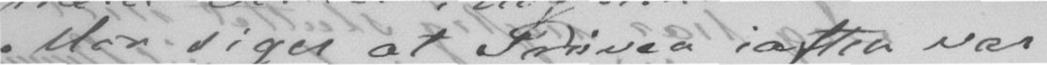Mor siger at Prøven iaften var
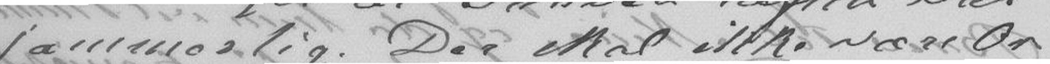jammerlig. Der skal ikke være Or-
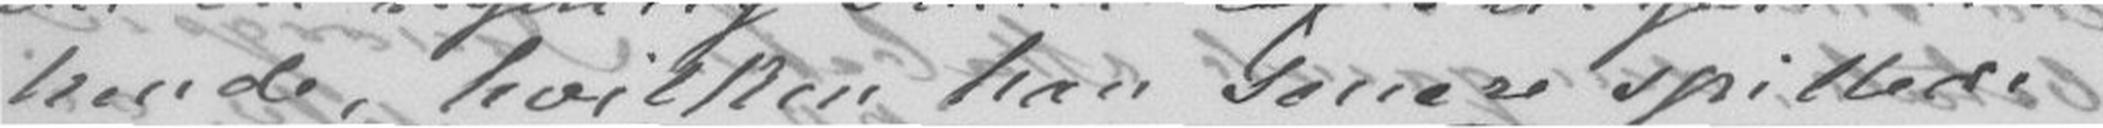hende, hvilken han senere spillede
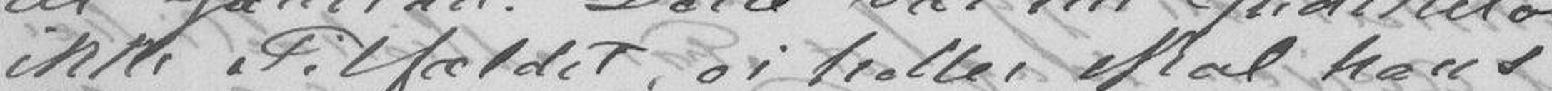ikke Tilfældet, ei heller skal hans
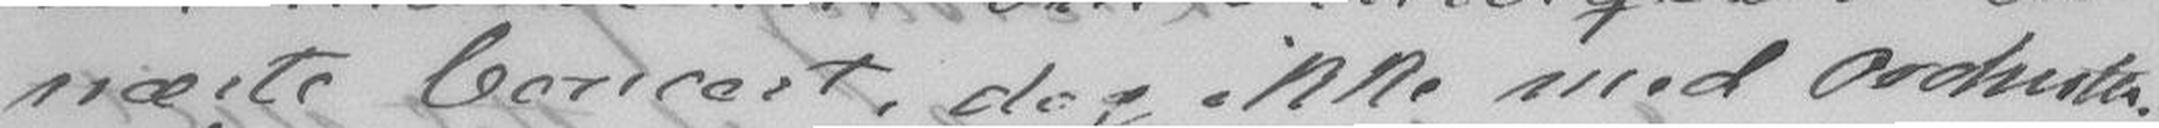næste Concert, dog ikke med Orchester.
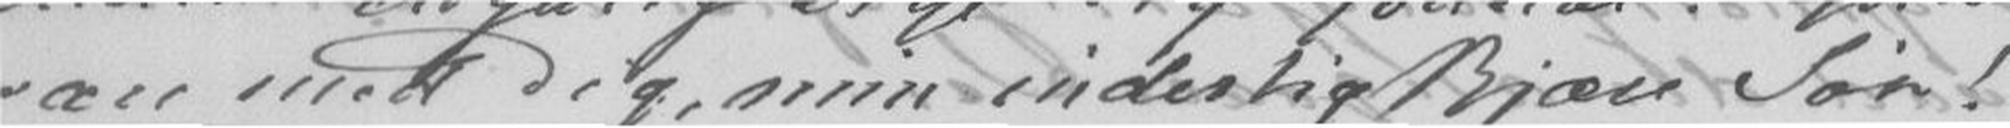være med Dig, min inderlig kjære Søn!
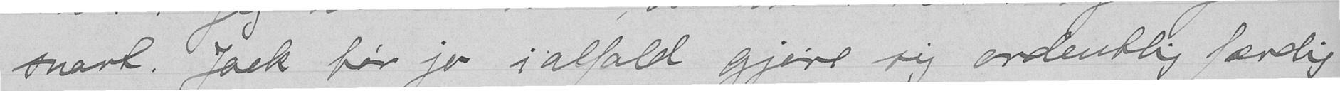snark. Jack bør jo ialfald gjøre sig ordentlig færdig
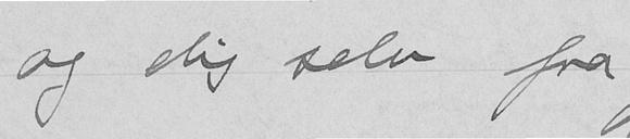og dig selv fra
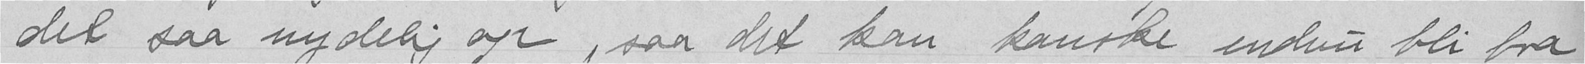det saa nydelig op, saa det kan kanske endnu bli bra
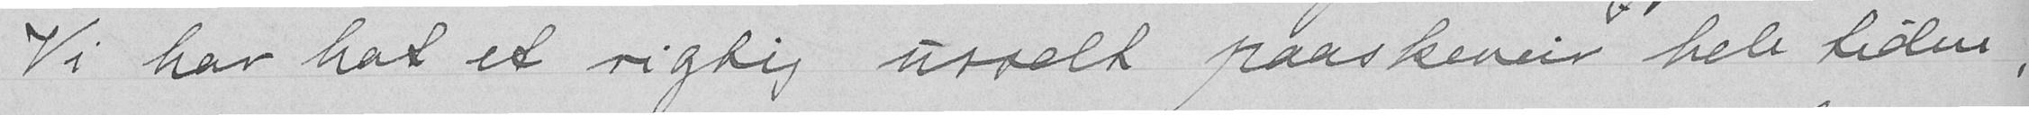Vi har har et rigtig usselt paaskeveir hele tiden,
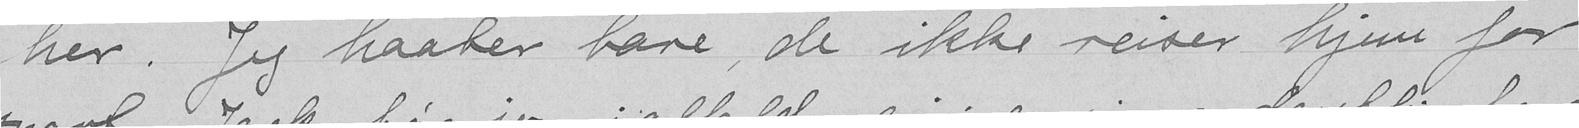her. Jeg haaber bare, de ikke reiser hjem for
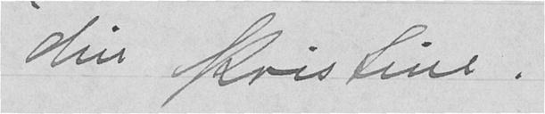din Kristine.
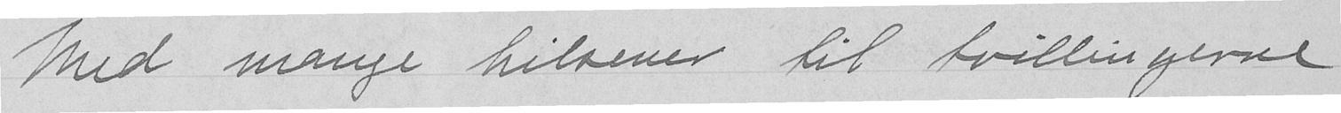Med mange hilsener til tvillingerne
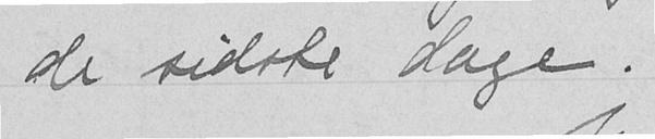de sidste dage.
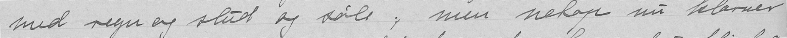med regn og slud og søle, men netop nu klarner
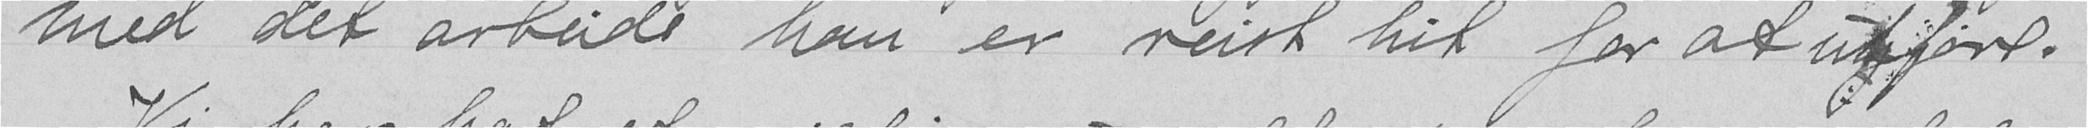med det arbeide han er reist hit for at utføre.
